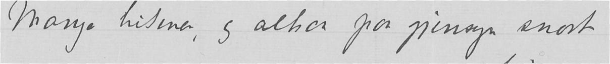Mange hilsener, og altsaa paa gjensyn snart
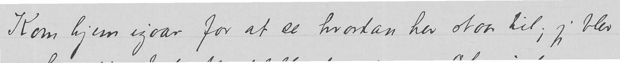Kom hjem igaar for at se hvordan her staar til; jeg blev
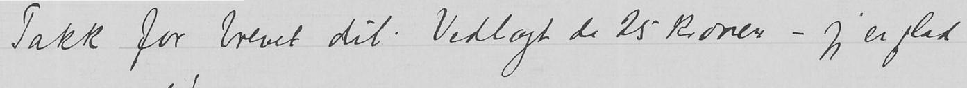Takk for brevet dit. Vedlagt de 25 kroner – jeg er glad
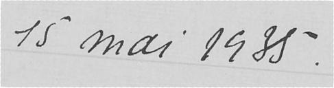15. mai 1935.
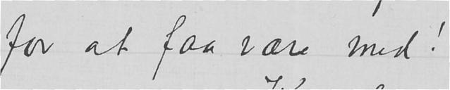for at faa være med!
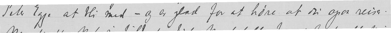Peter Egge at bli med – og er glad for at høre at du ogsaa reiser.
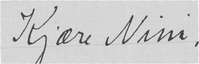Kjære Nini
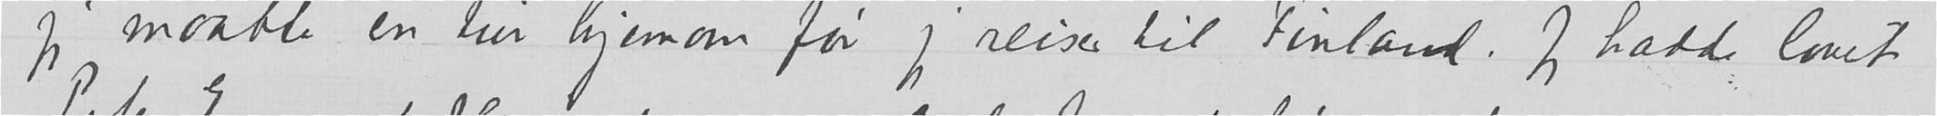jeg maatte en tur hjemom før jeg reiser til Finland. Jeg hadde lovet
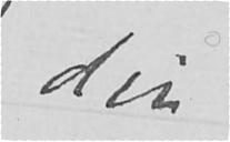din
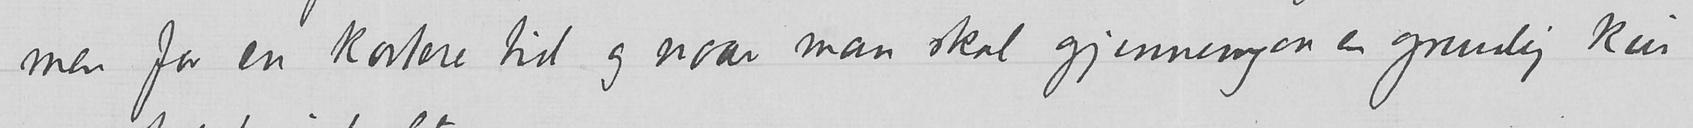men for en kortere tid og naar man skal gjennemgaa en grundig kur
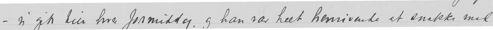– vi gik tur hver formiddag, og han var helt henrivende at snakke med
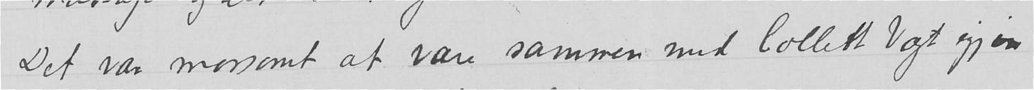Det var morsomt at være sammen med Collett Vogt igaar
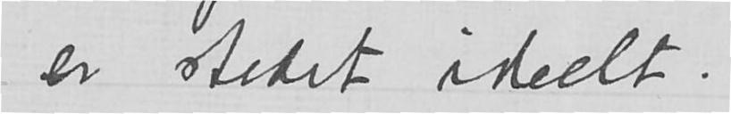er stedet ideelt.
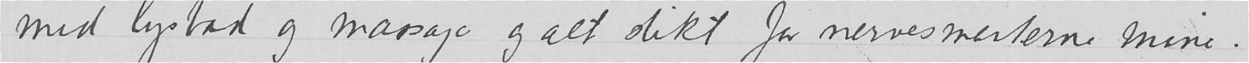med lysbad og massage og alt slikt for nervesmerterne mine.
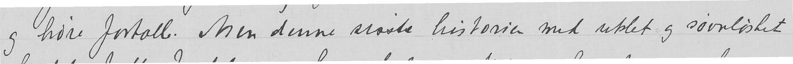og høre fortelle. Men denne sisste historien med utlet og søvnløstet
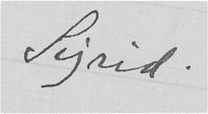Sigrid
